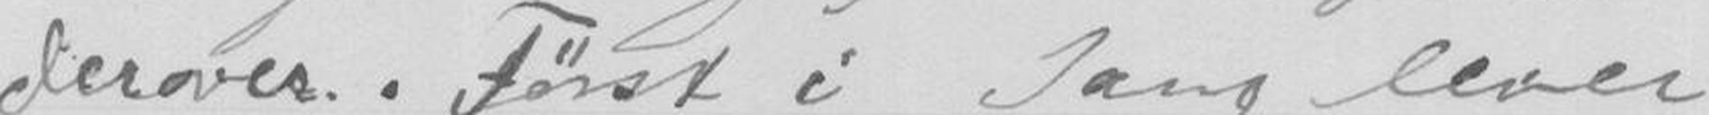derover. Først i Sang lever
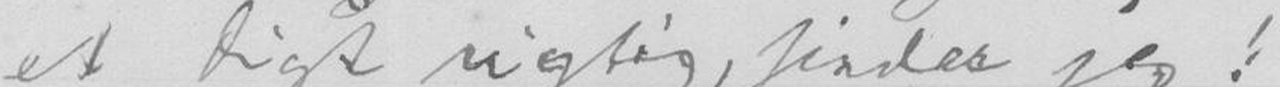et Digt rigtig, finder jeg!
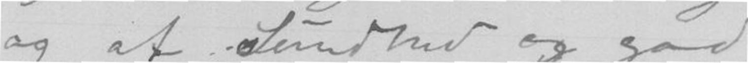og at Sundhed og god
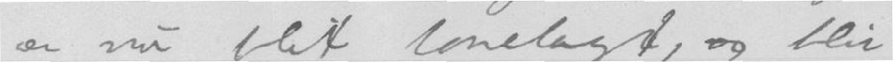er nu blit tonelagt, og blir
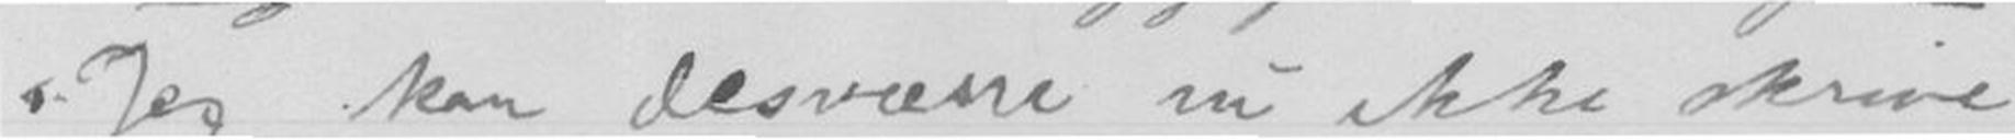Jeg kan desværre nu ikke skrive
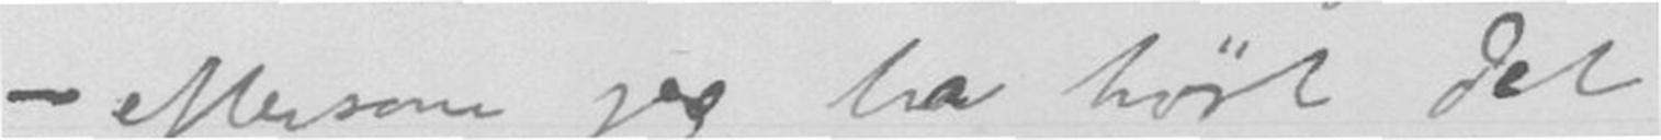- eftersom jeg har hørt det
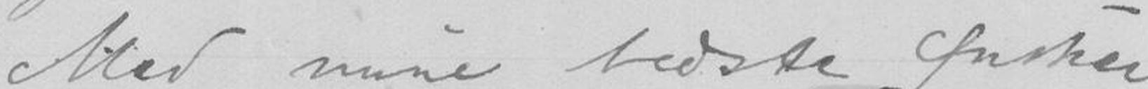Med mine bedste Ønsker,
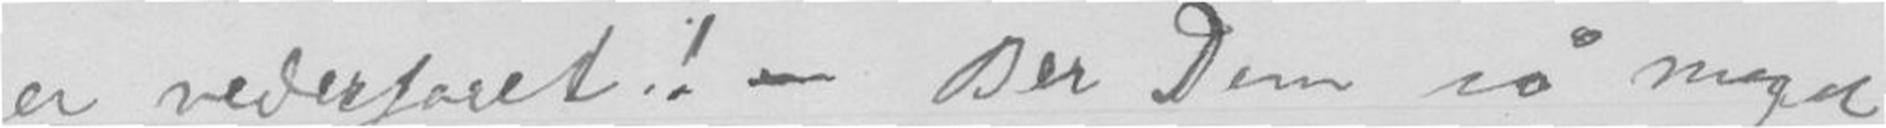er vederfaret! - Ber Dem så meget
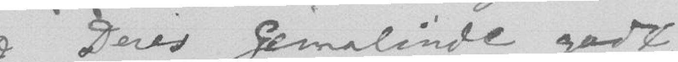og Deres Gemalinde godt,
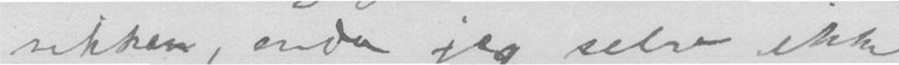sikken, enda jeg selv ikke
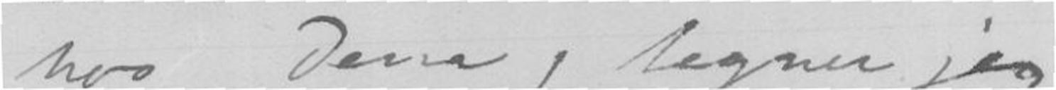hos Dem, tegner jeg,
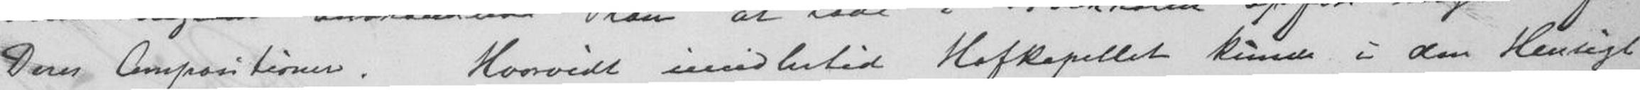Deres Compositioner. Hvorvidt imidlertid Hofkapellet kunde i den Henligt
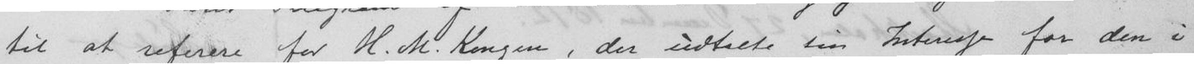til at referere for H.K. Kongen, der udtalte sin Interesje for den i
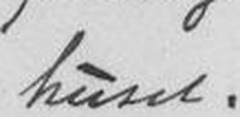huset.
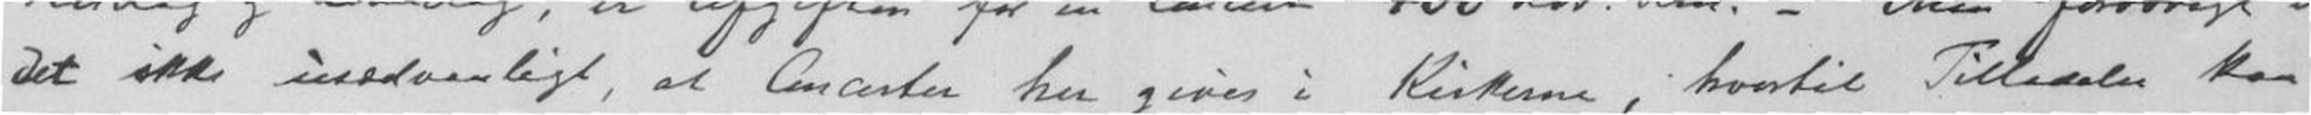det ikke usædvanligt, at Concerter her gives i Kirkerne, hvortil Tilladelse kan
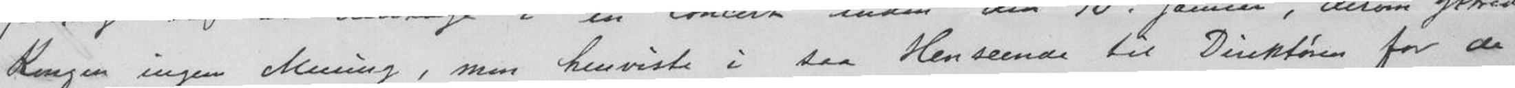Kongen ingen Mening, men herviste i saa Henseende til Direktøren for de
